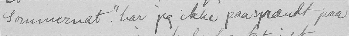Sommernat", har jeg ikke paaspændt paa
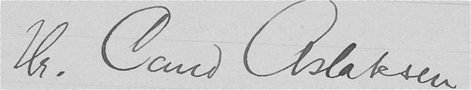Hr. Cand. Aslaksen
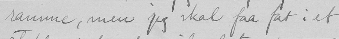ramme; men jeg skal faa fat i et
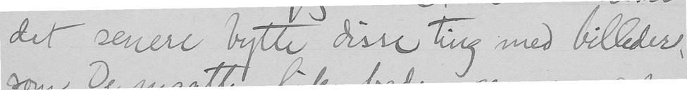det senere bytte disse ting med billeder,
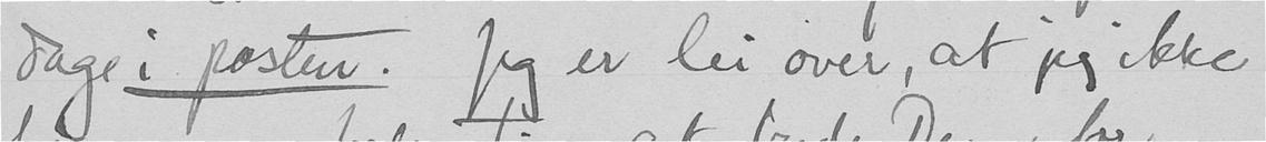dage i posten. Jeg er lei over, at jeg ikke
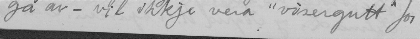gå av – vil ikkje vera "visergutt" for
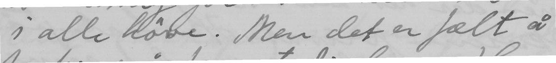i alle höve. Men det er fælt å
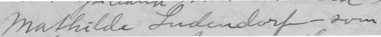Mathilde Ludendorf – som
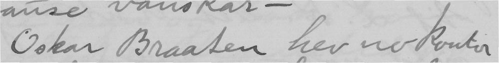Oskar Braaten hev no kontor
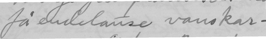få endelause vanskar –
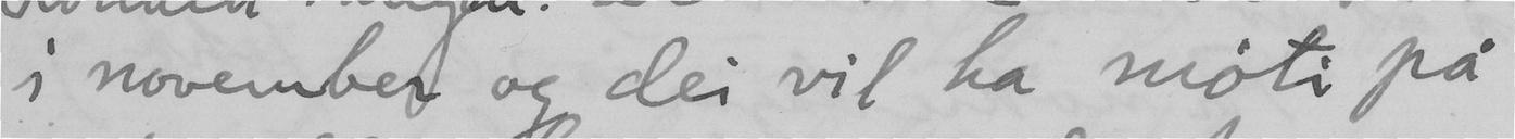i november og dei vil ha möti på
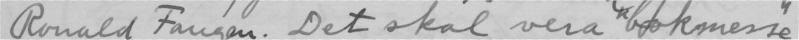Ronald Fangen. Det skal vera "bokmesse"
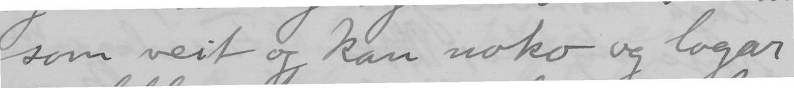som veit og kan noko og logar
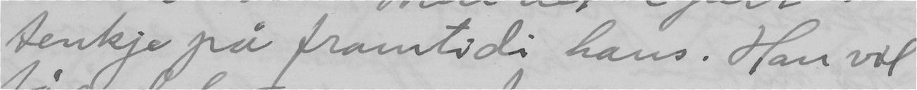tenkje på framtidi hans. Han vil
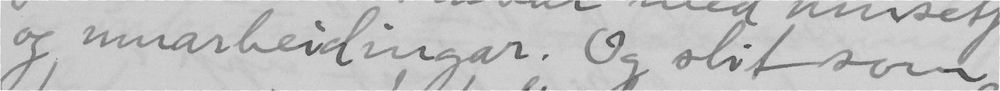og umarbeidingar. Og slit som
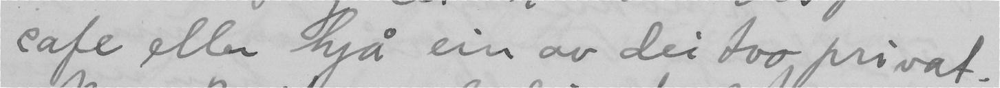cafe eller hjå ein av dei tvo privat.
